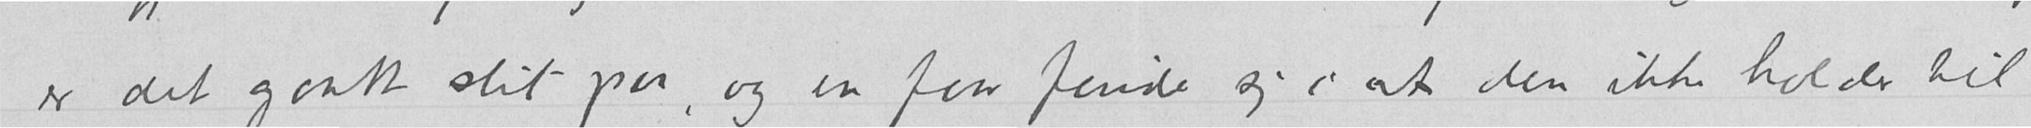er det gaatt slit paa, og en faar finde sig i at den ikke holder til
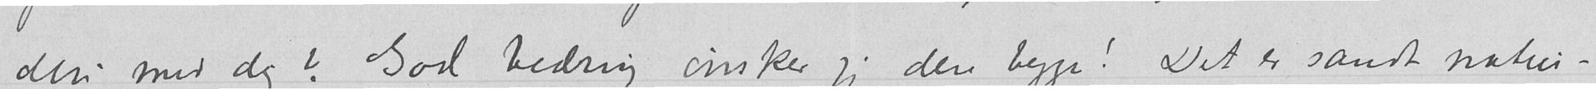din med dig? God bedring ønsker jeg dere begge! Det er sandt natur-
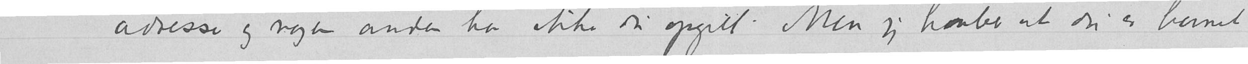adresse og nogen anden har ikke du opgitt. Men jeg haaber at du er havnet
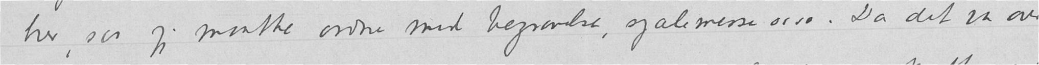her, saa jeg maatte ordne med begravelse, sjælemesse osv. Da det var over
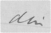din
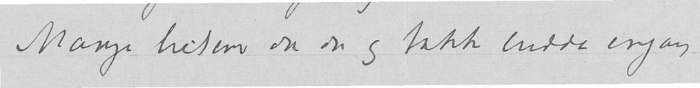Mange hilsener da du og takk endda engang
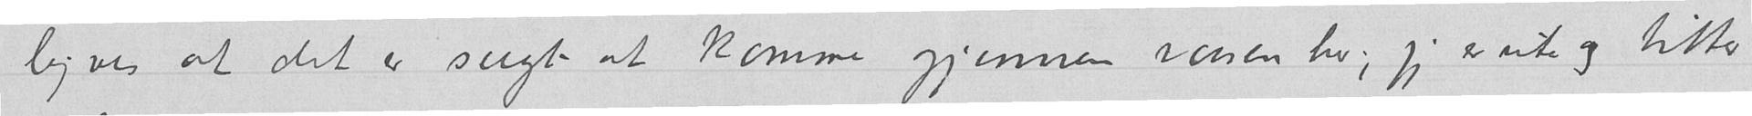ligvis at det er seigt at komme gjennem vaaren her; jeg er ute og titter
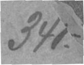341.
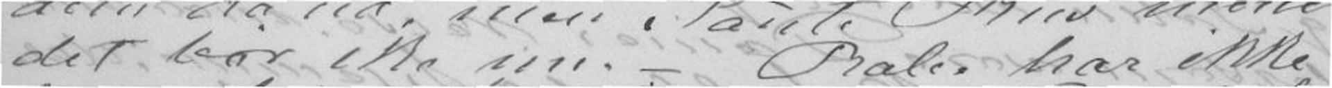det bør ske nu. - Rabe har ikke
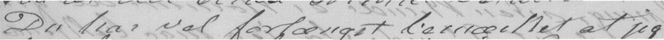Du har vel forlængst bemærket at jeg
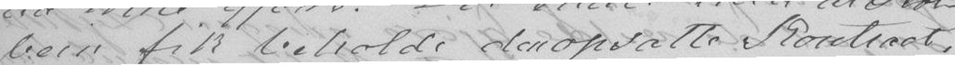bein fik beholde den opsatte Kontract,
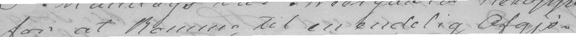for at komme til en endelig Afgjø-
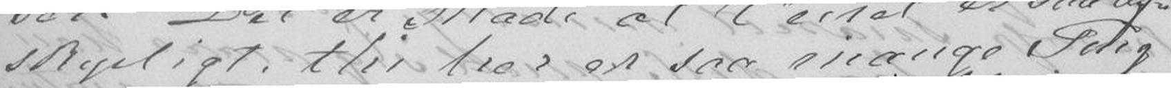skyligt, thi her er saa mange Ting
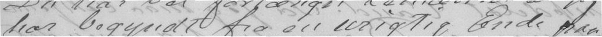har begyndt fra en urigtig Ende paa
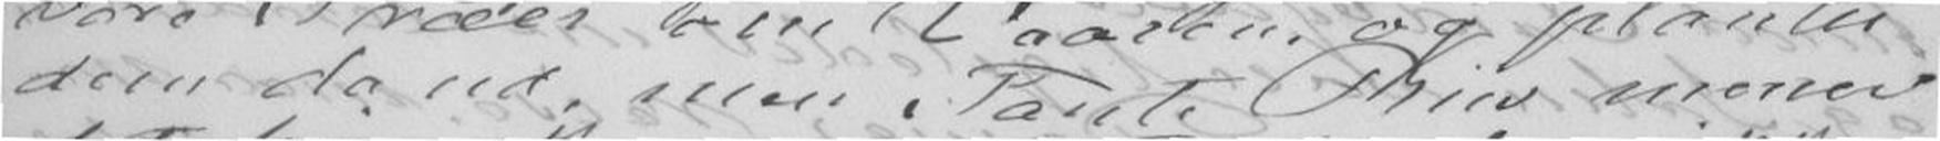dem da ud, men Tante Riis mener
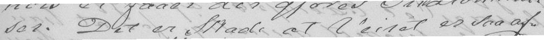ser. Det er Skade at Veiret er saa af-
